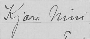Kjære Nini,
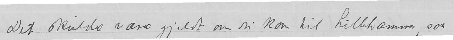Det skulde være gjildt om du kom til Lillehammer, saa
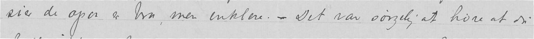sier de ogaa er bra, men enklere. – Det var sørgelig at høre at du
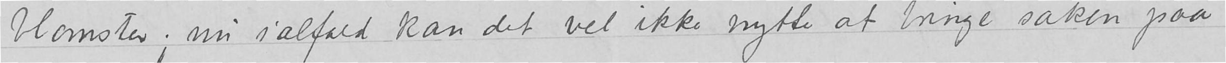blomster; nu ialfald kan det vel ikke nytte at bringe saken paa
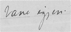bane igjen.
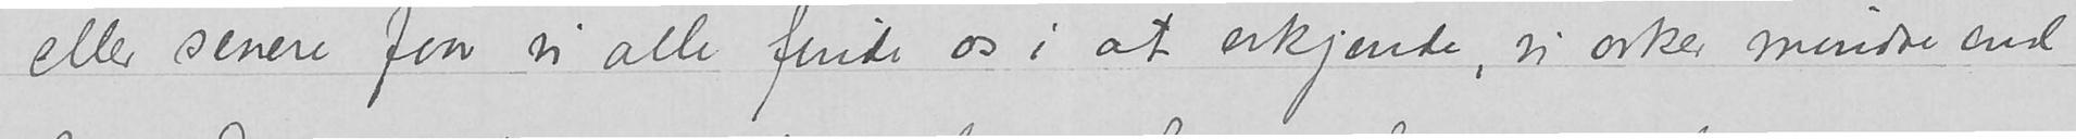eller senere faar vi alle finde os i at erkjende, vi orker mindre end
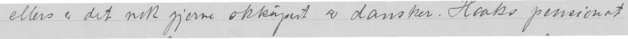ellers er det nok gjerne okkupert av dansker. Haaks pensionat
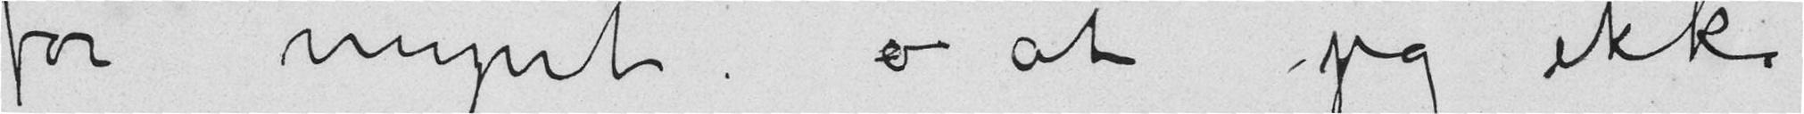for mynt at jeg ikke
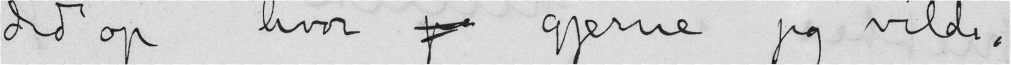didop hvor je gjerne jeg vilde.
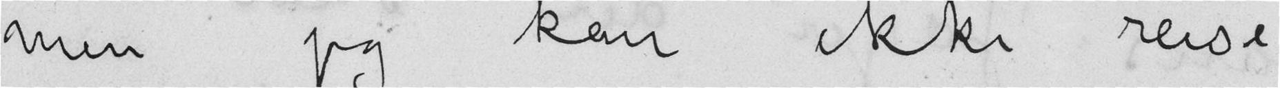men jeg kan ikke reise
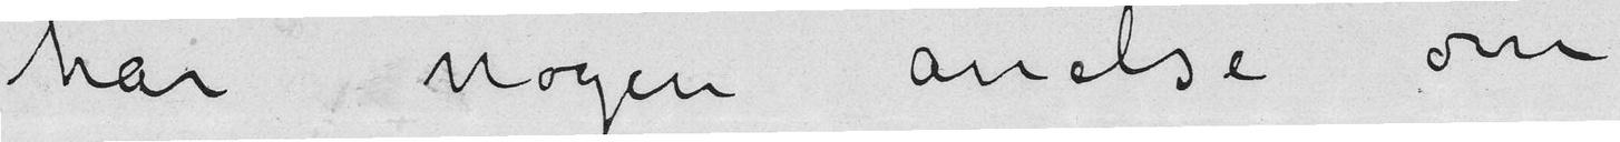har nogen anelse om
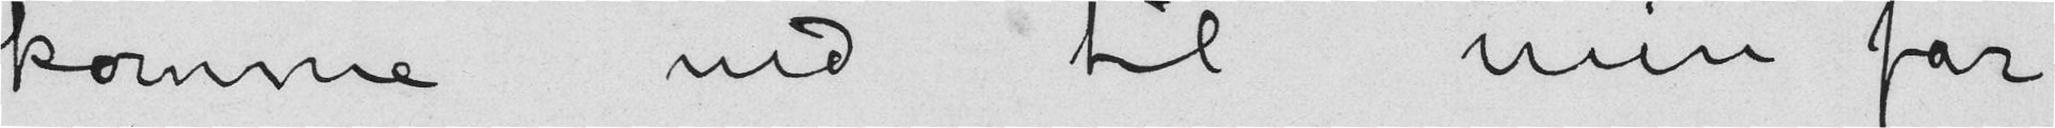komme ned til min far
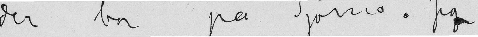der bor på Tjømø. Jeg
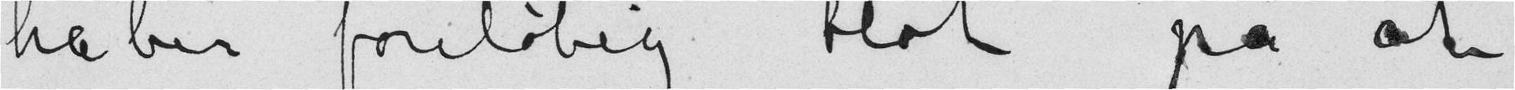håber foreløbig blot på at
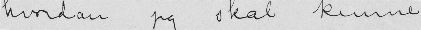hvordan jeg skal kunne
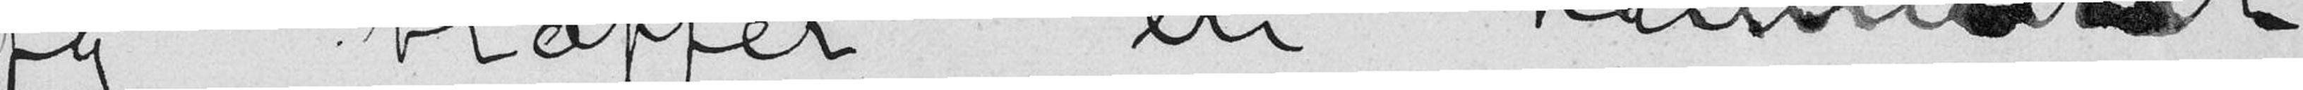jeg træffer en kammerat
